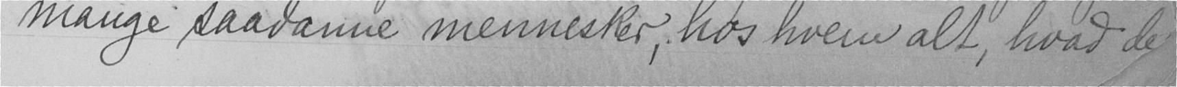mange saadanne mennesker, hos hvem alt, hvad de
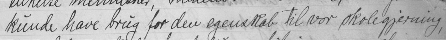kunde have brug for den egenskab til vor skolegjerning,
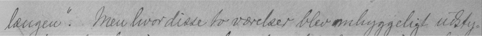længen". Men hvor disse to værelser blev omhyggeligt udsty-
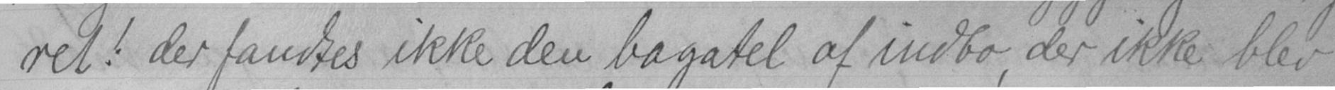ret! der fandtes ikke den bagatel af indbo, der ikke blev
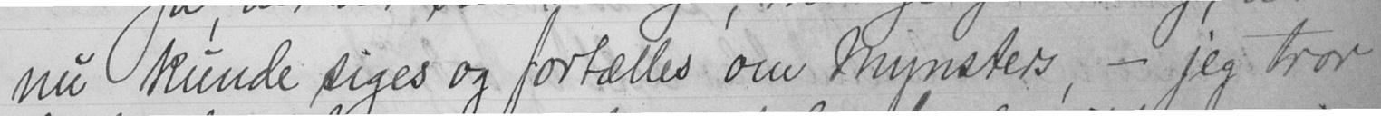nu, kunde siges og fortælles om Mynsters, - jeg tror
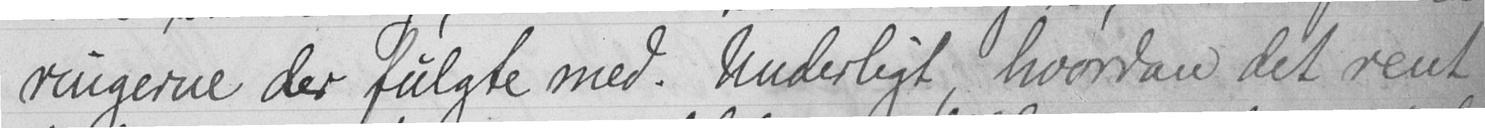ringerne der fulgte med. Underligt, hvordan det rent
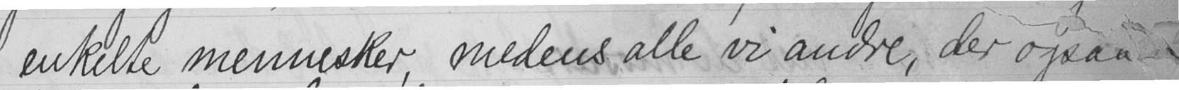enkelte mennesker, medens alle vi andre, der ogsaa -
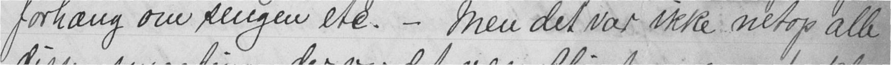forhæng om sengen etc. - men det var ikke netop alle
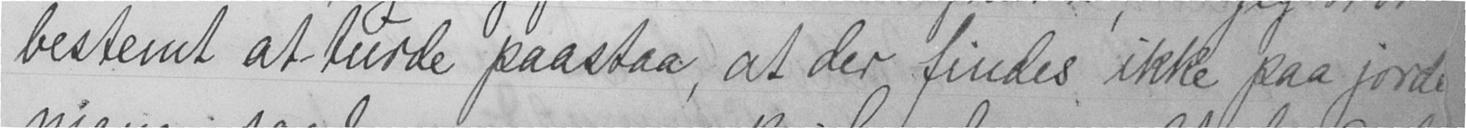bestemt at turde paastaa, at der findes ikke paa jorden
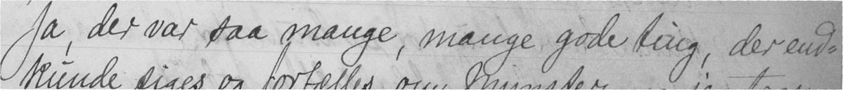Ja, der var saa mange, mange gode ting, der end-
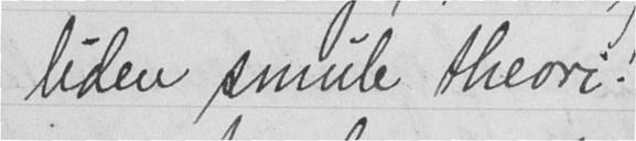liden smule theori!
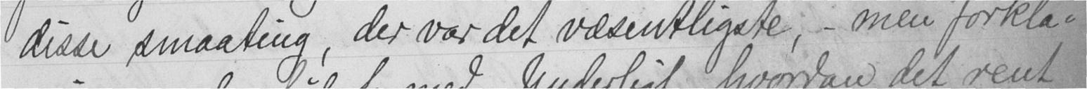disse smaating, der var det væsentligste, - men forkla-
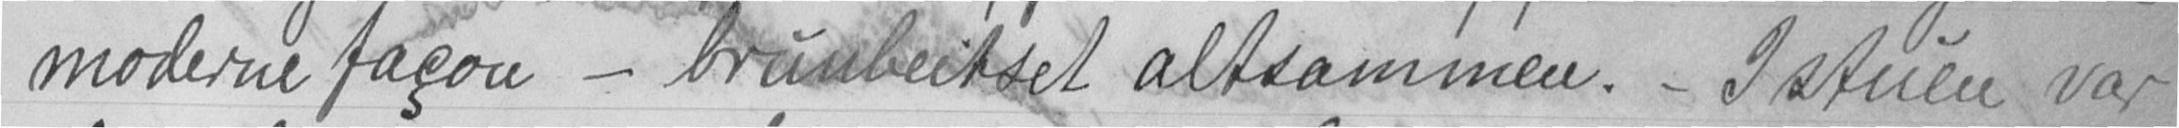moderne façon - brunbeitset altsammen. - I stuen var
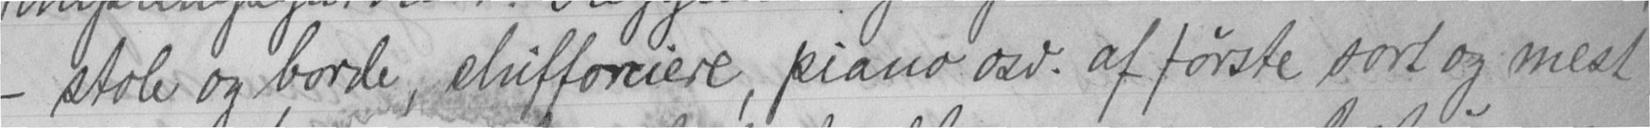- stole og borde, chiffoniere, piano osv. af første sort og mest
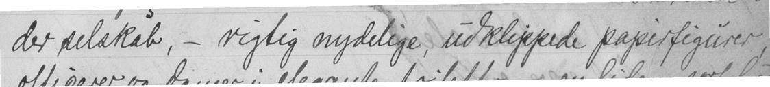der selskab, - rigtig nydelige, udklippede papirfigurer,
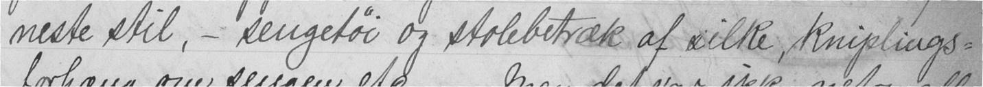neste stil, - sengetøi og stolebetræk af silke, kniplings-
